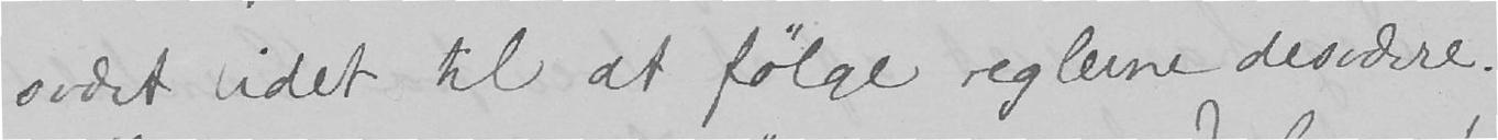svært lidet til at følge reglerne desværre.
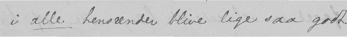i alle henseender blive lige saa godt.
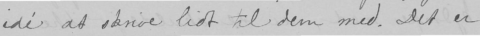idé at skrive lidt til dem med. Det er
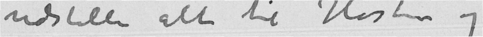udstille alt til Høsten og
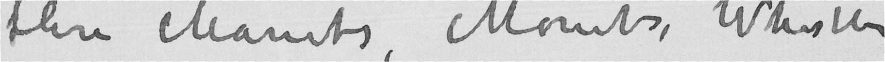flere Manets, Monets, Whistler og
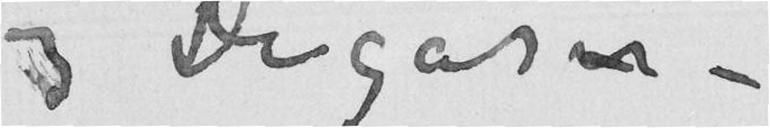Degaser –
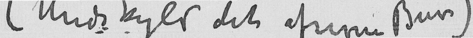(Undskyld det afrevne Brev)
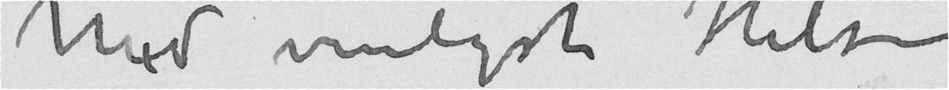Med venligste Hilsen
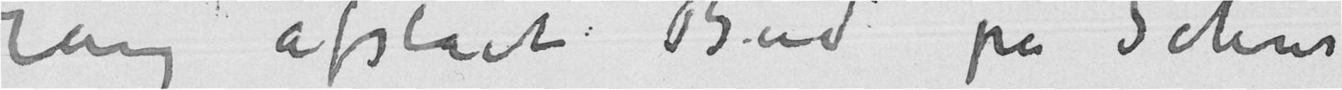Gang afslået Bud på Schous
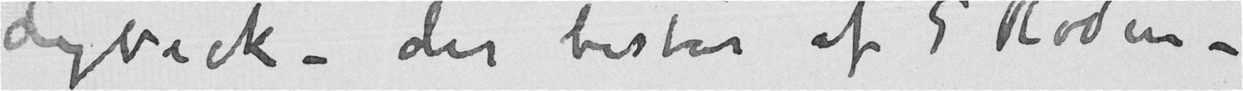Lybeck – der består af 5 Rodin –
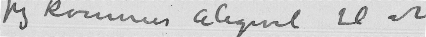jeg kommer aligevel til at
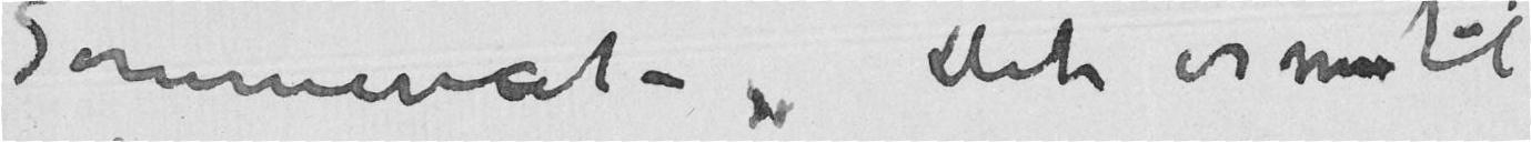Sommernat – Det er nu til
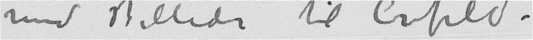med Billeder til Crefeld.
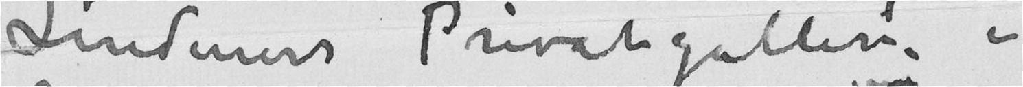Lindeners Privatgalleri i
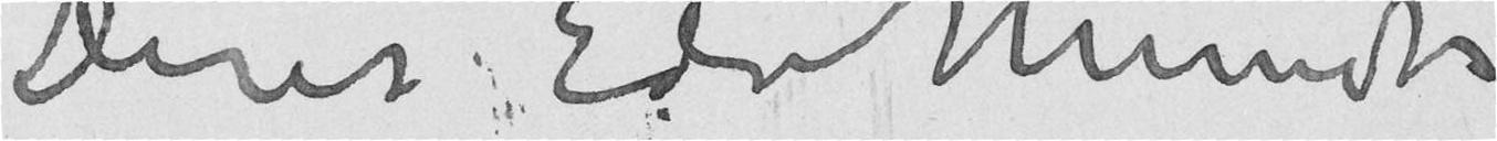Deres Edv Munch
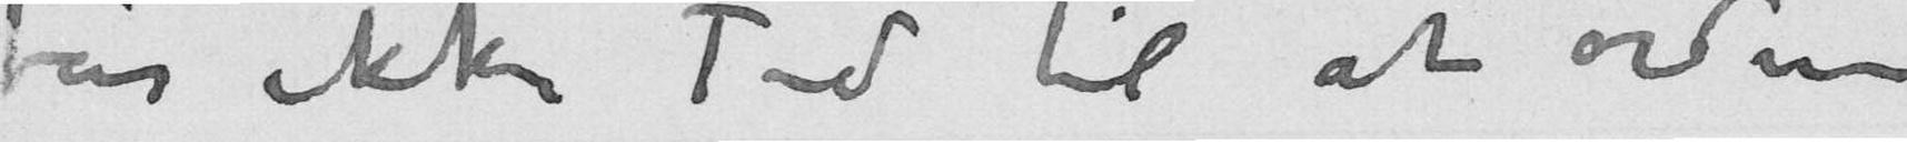får ikke Tid til at ordne
